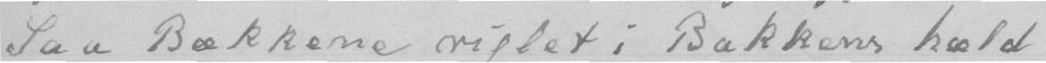Saa Bækkene vislet i Bakkens hæld
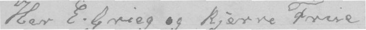Her E. Grieg og kjerre Frue
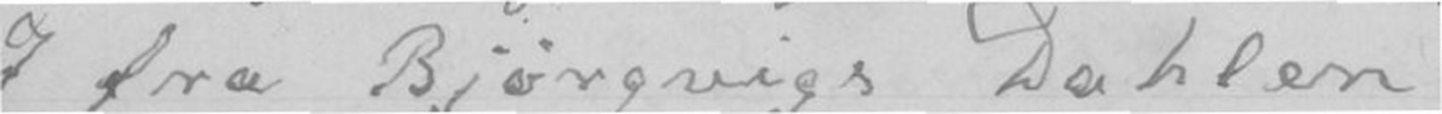I fra Bjørgvigs Dahlen
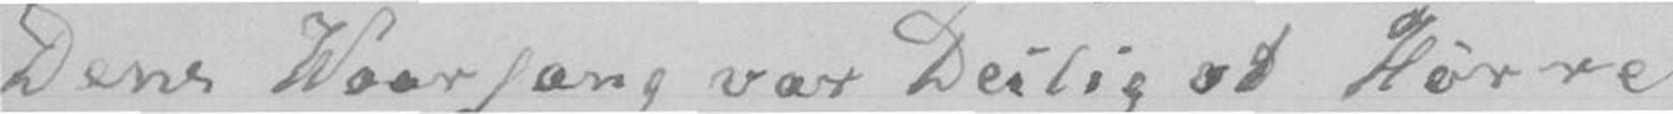Dens Waargang var Deilig at Hørre
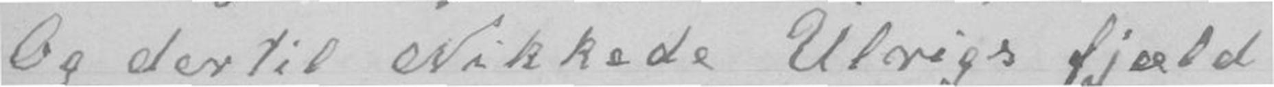Og dertil Nikkede Ulrigs fjæld
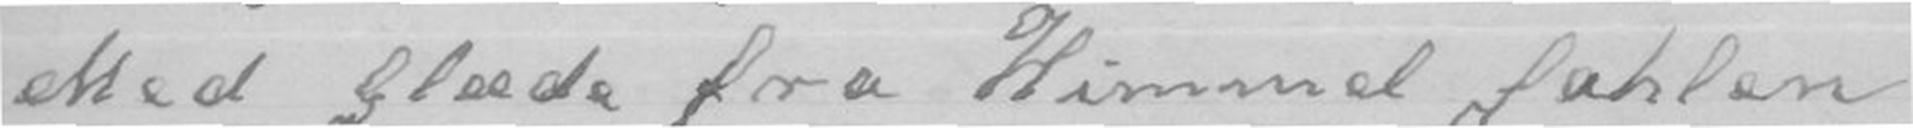Med glæde fra Himmel Sahlen
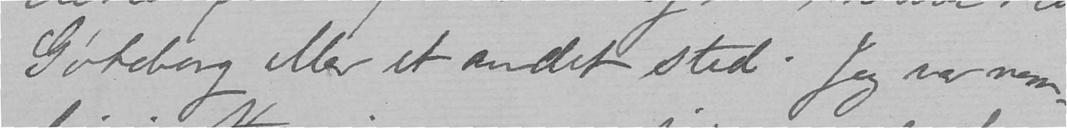Gøteborg eller et andet sted. Jeg var nemm-
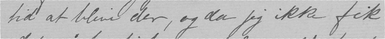tid at blive der, og da jeg ikke fik
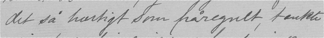det så hurtigt som påregnet, tænkte
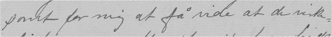somt for mig at få vide at de virke-
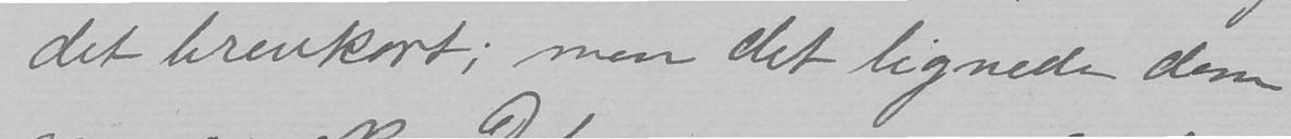det brevkort; men det lignede dem
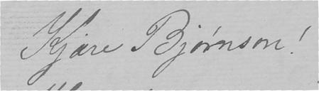Kjære Bjørnson!
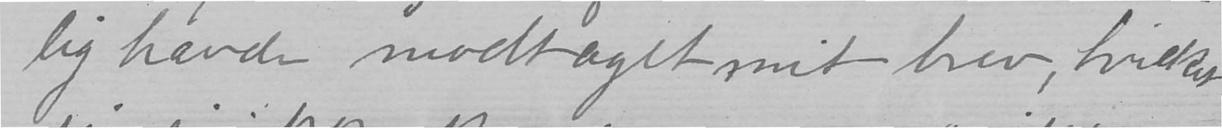lig havde modtaget mit brev hvilket
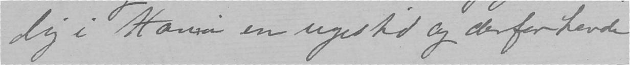elig i Xania en uges tid og derfor havde
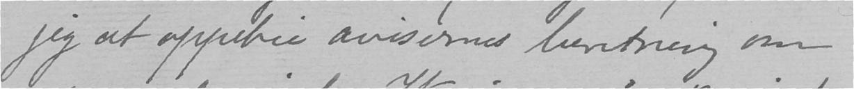jeg at oppebie avisernes beretning om
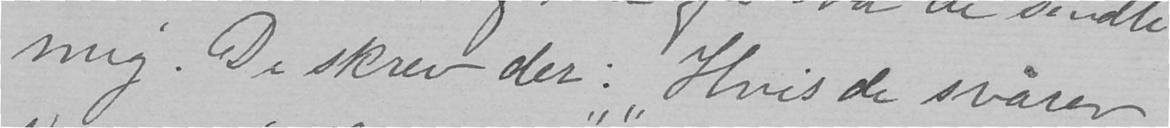mig. De skrev der: "Hvis de svarer
